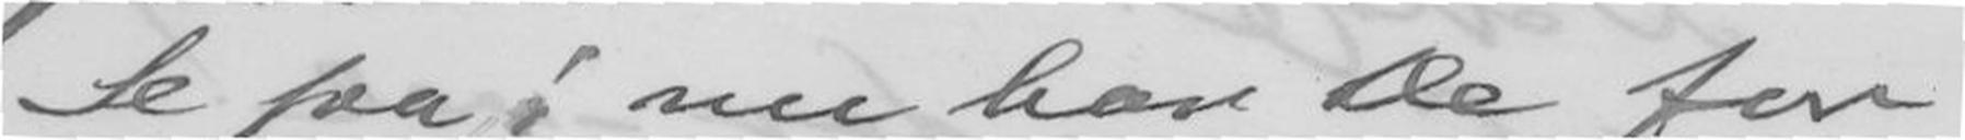Se saa! nu har De for
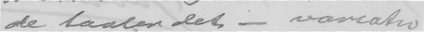de taaler det - vancatro
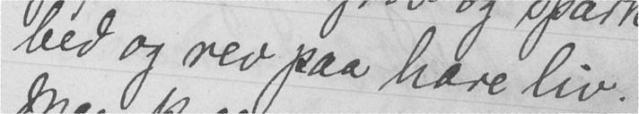leid og rev paa hare liv.
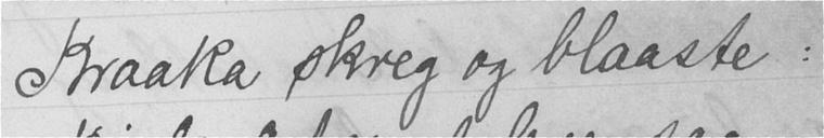Kraaka skreg og blaaste:
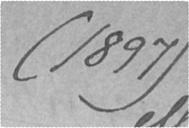(1897)
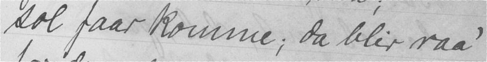sol faar komme; da blir raa'
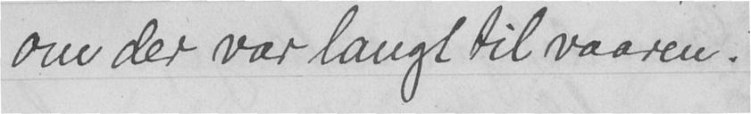om der var langt til vaaren.
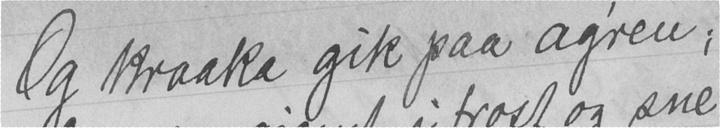Og kraaka gik paa ag'ren;
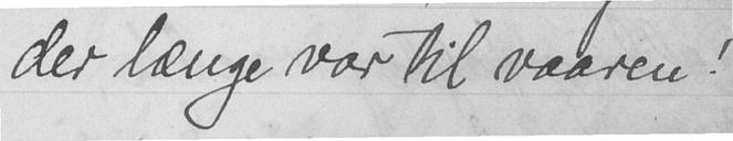der længe var til vaaren!
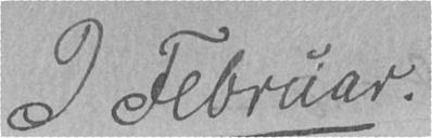I Februar.
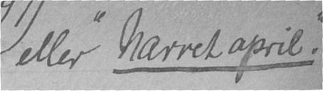eller "Narret april."
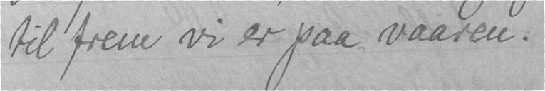til frem vi er paa vaaren!
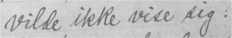vilde ikke vise sig:
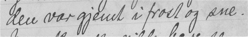den var gjemt i frost og sne.
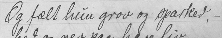Og fælt hun grov og sparked, -
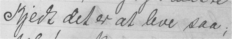Kjedt det er at leve saa;
